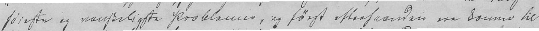høieste og vanskeligste Problemer, og først efterhaanden ere komne til
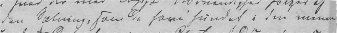den Sætning, som de have fundet i den menne-
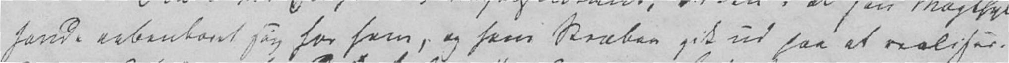havde aabenbaret sig for ham, og hans Stræben gik ud paa at realisere
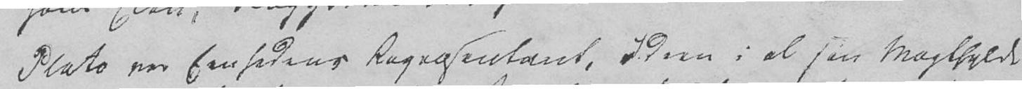Plato var Eenhedens Repræsentant, Ideen i al sin Magtfylde
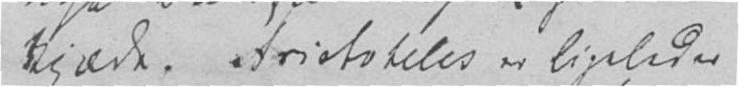kjæde. Aristoteles er ligeledes
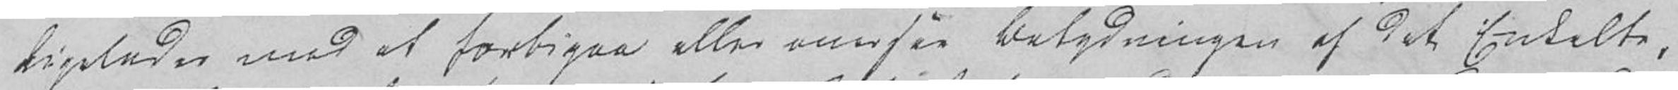ligeledes med at forbigaa eller oversee Betydningen af det Enkelte,
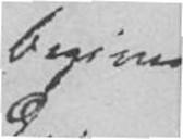Begiven-
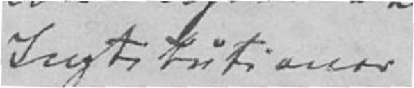Institutioner
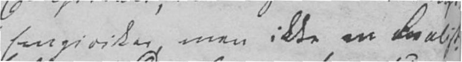Empiriker, men ikke en Realist.
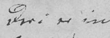Deri er imid-
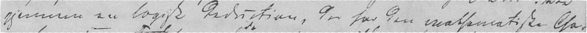gjennem en logisk Deduction, der har den mathematiske Cha-
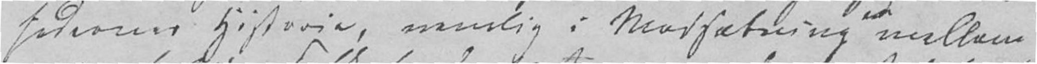hedernes Historie, nemlig i Modsætning mellem
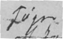søger
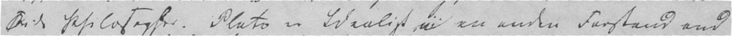Tids Philosopher. Plato er Idealist i en anden Forstand end
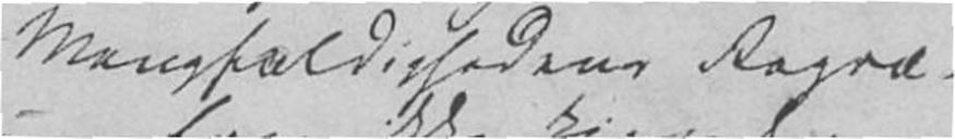Mangfoldighedens Repræ-
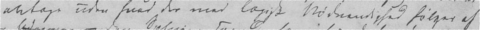antage uden hvad der med logisk Nødvendighed følger af
